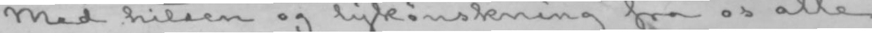Med hilsen og lykønskning fra os alle
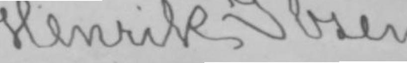Henrik Ibsen.
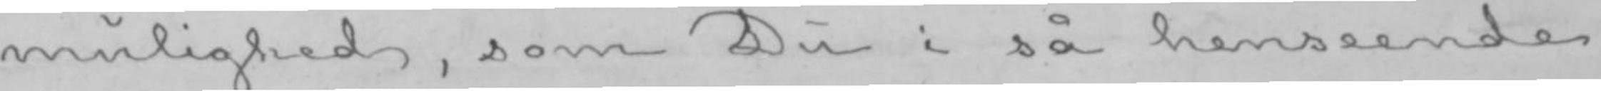mulighed, som Du i så henseende
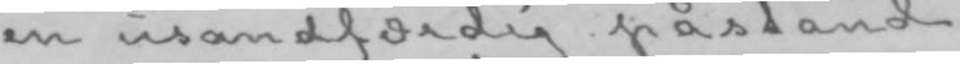en usandfærdig påstand.
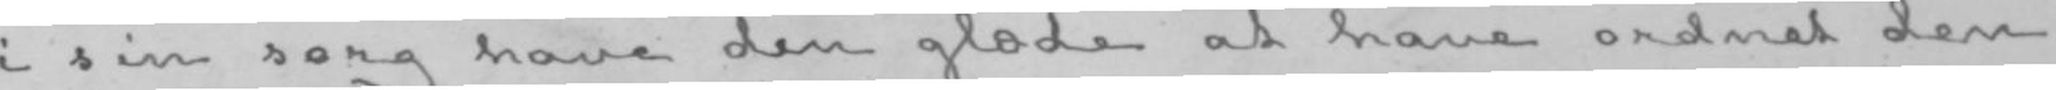i sin sorg have den glæde at have ordnet den
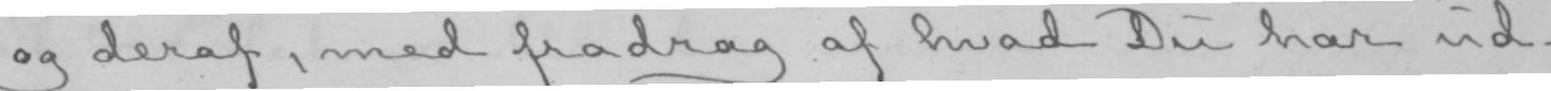og deraf, med fradrag af hvad Du har ud-
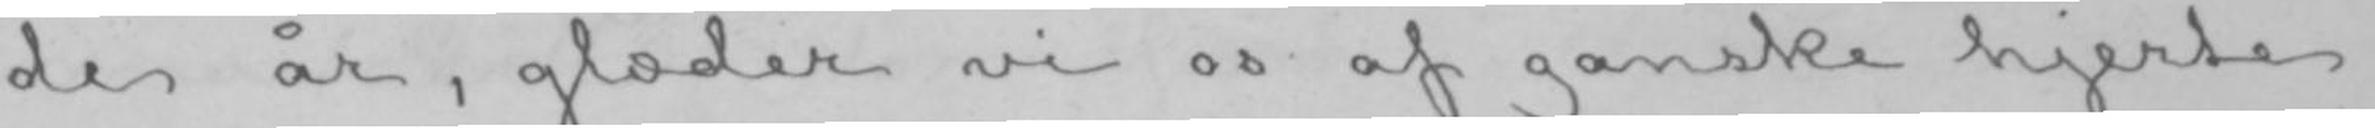de år, glæder vi os af ganske hjerte
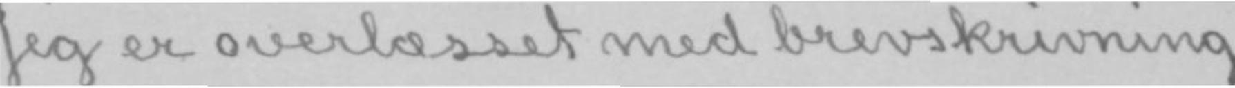Jeg er overlæsset med brevskrivning
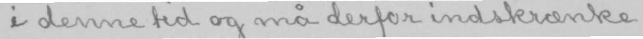i denne tid og må derfor indskrænke
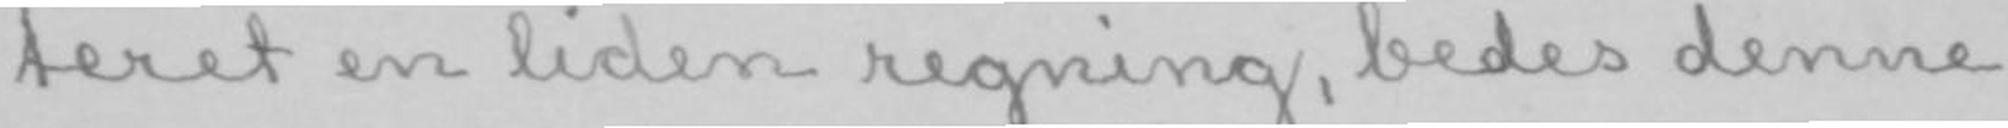teret en liden regning, bedes denne
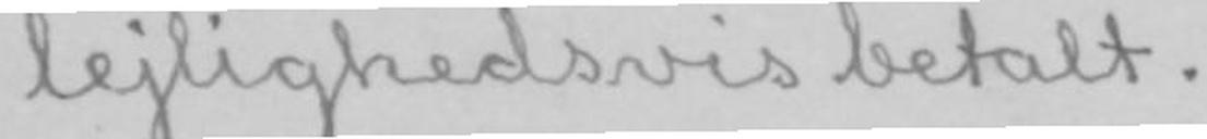lejlighedsvis betalt.
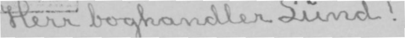Herr boghandler Lund!
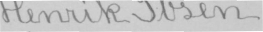Henrik Ibsen.
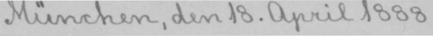München, den 18. April 1888.
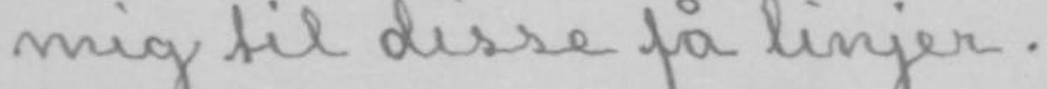mig til disse få linjer.
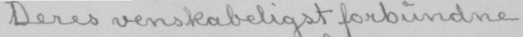Deres venskabeligst forbundne
